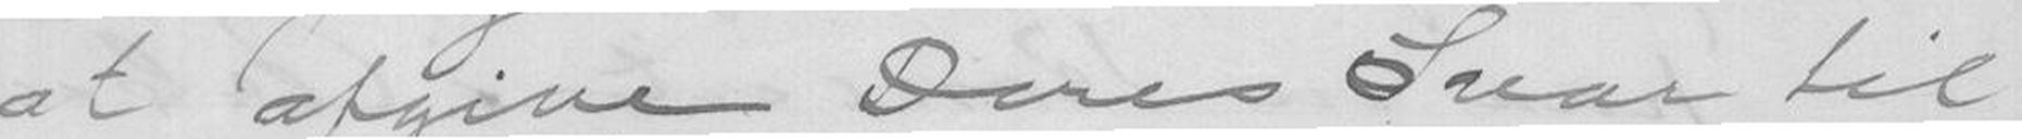at afgive Deres Svar til
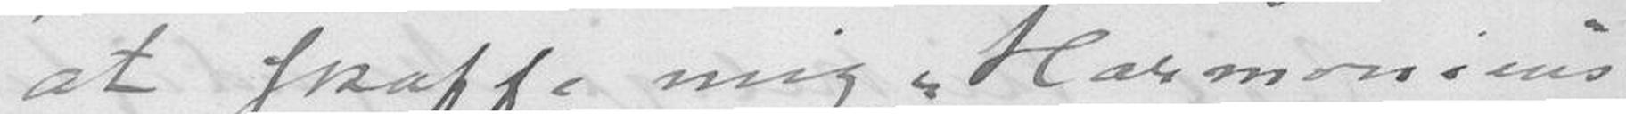at skaffe mig "Harmoniens"
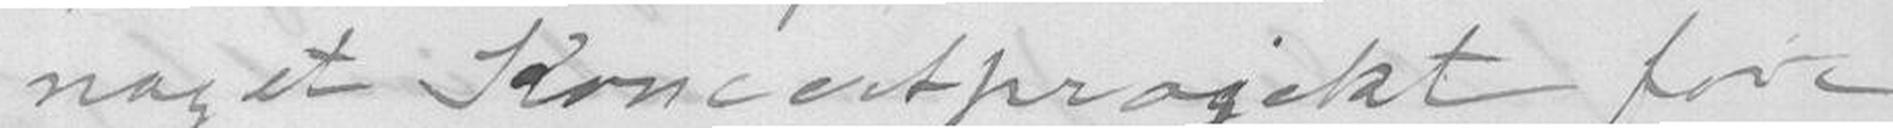noget Koncertprosjekt føre
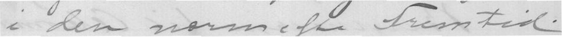i den nærmeste Fremtid.
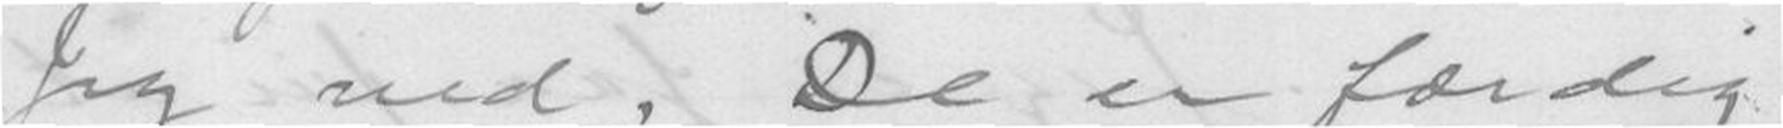Jeg ved, De er færdig
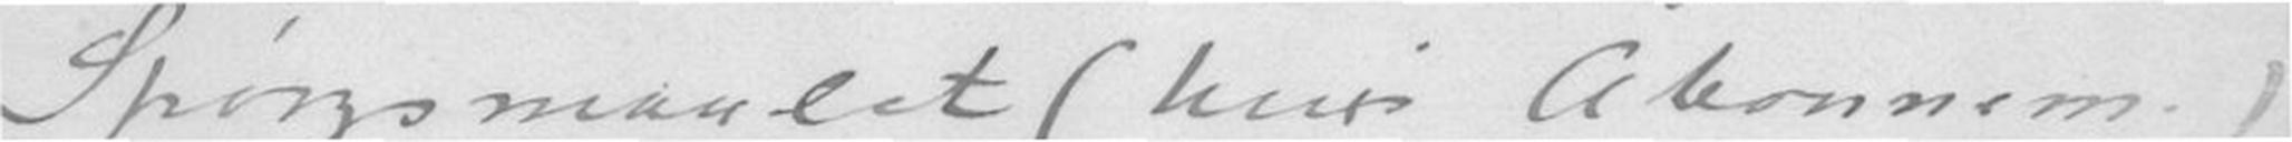Spørgsmaalet (hvis Abonnem.)
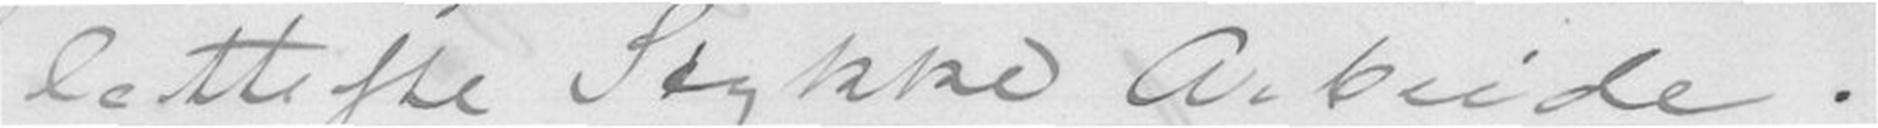letteste Stykke Arbeide.
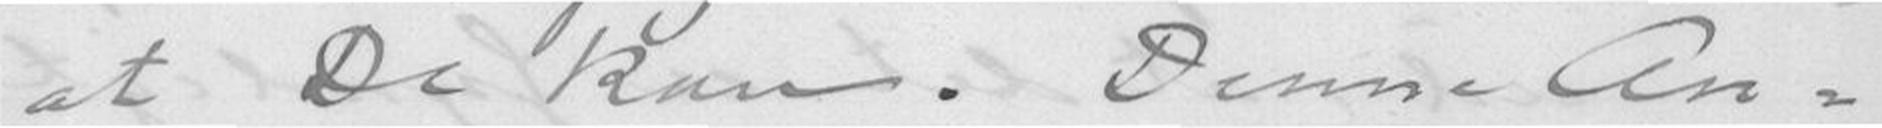at De kan. Denne An-
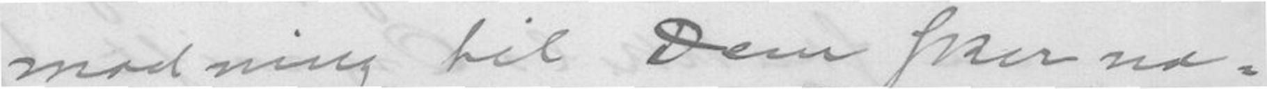modning til Dem sker na-
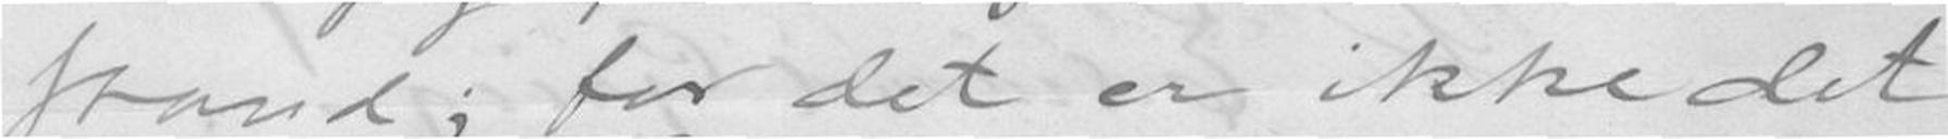stand; for det er ikke det
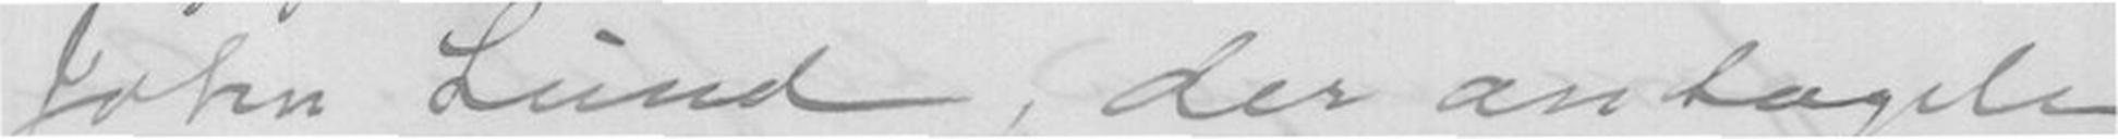John Lund, der antagelig
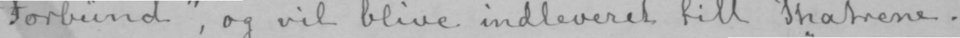Forbund», og vil blive indleveret till Thatrene.
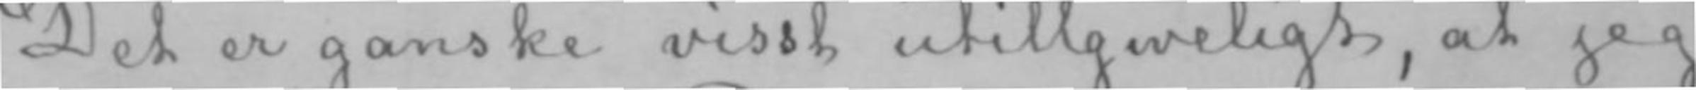Det er ganske visst utillgiveligt, at jeg
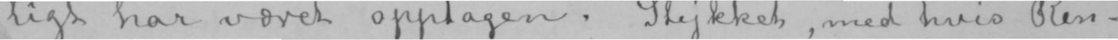ligt har været opptagen. Stykket, med hvis Ren-
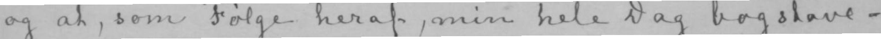og at, som Følge heraf, min hele Dag bogstave-
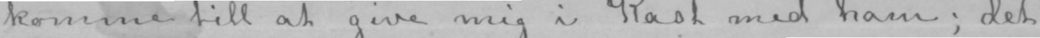komme till at give mig i Kast med ham; det
